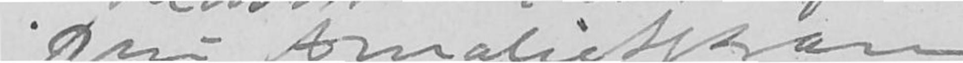Din Amalie Skram
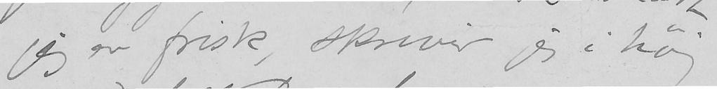jeg er frisk. Skriver jeg i høj
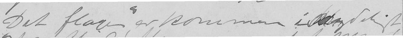"Det flager" er kommen i nydeligt
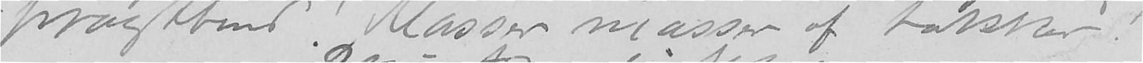pragtbind! Masser masser af takker!
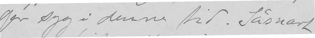ger syg i denne tid. Såsnart
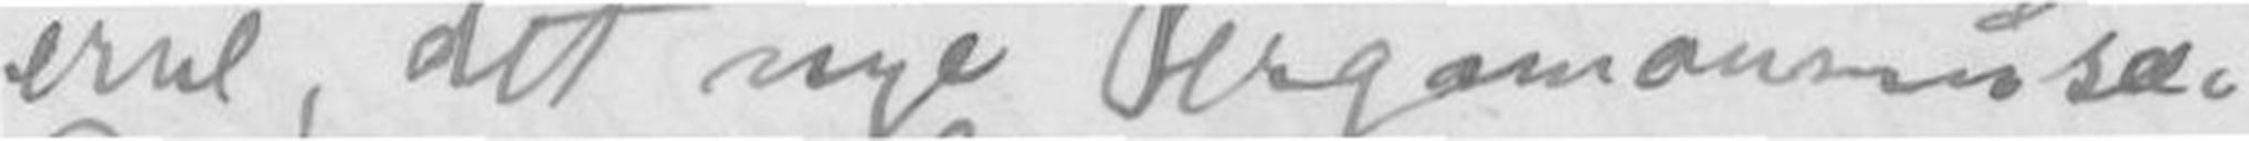erne, det rige Pergamonsmuse-
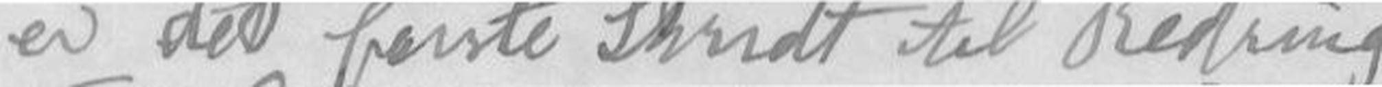er det første Skridt til Bedring
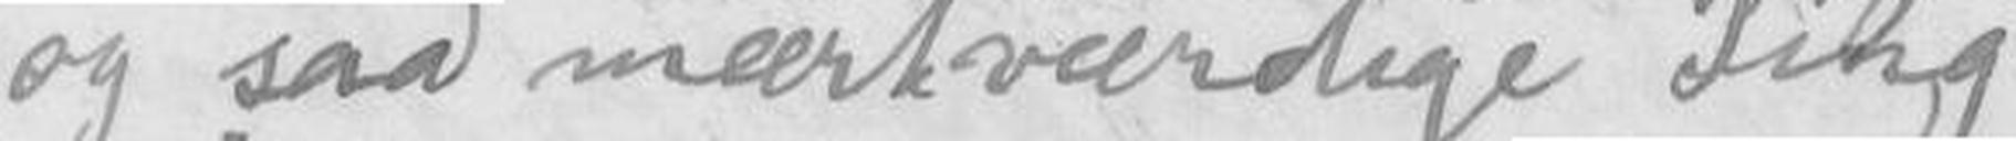og saa mærkværdige Ting.
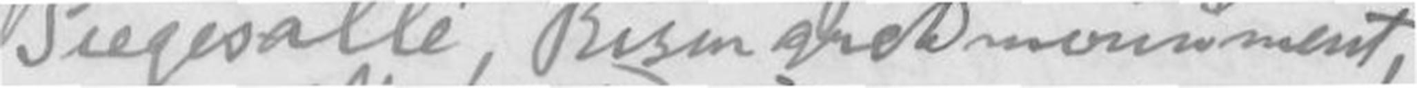Siegesallè, Bisengrekmonument,
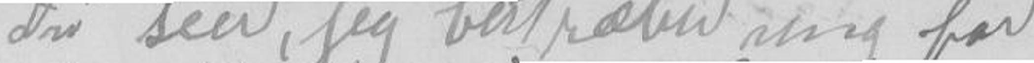Du seer, jeg bestræber mig for
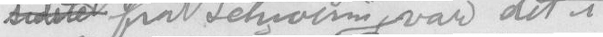fra Schwerin var det i
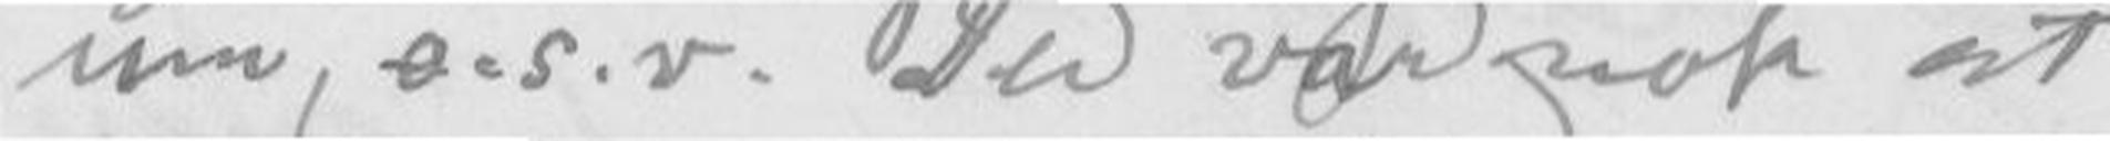um, o. s. v. Der var nok at
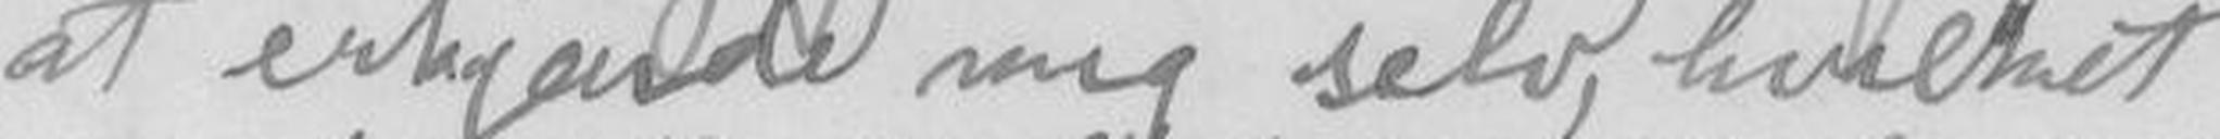at erkjænde mig selv, hvilket
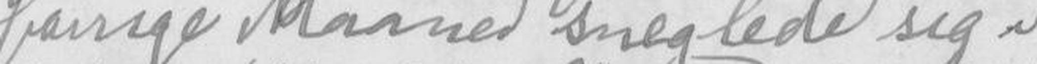forrige Maaned sneglede sig i
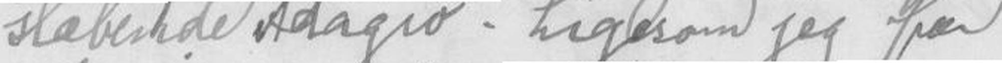slæbende Adagio. Ligesom jeg for
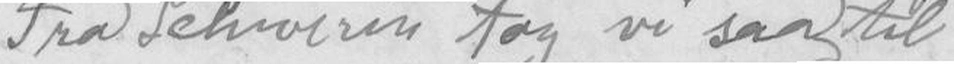Fra Schwerin tog vi saa til
